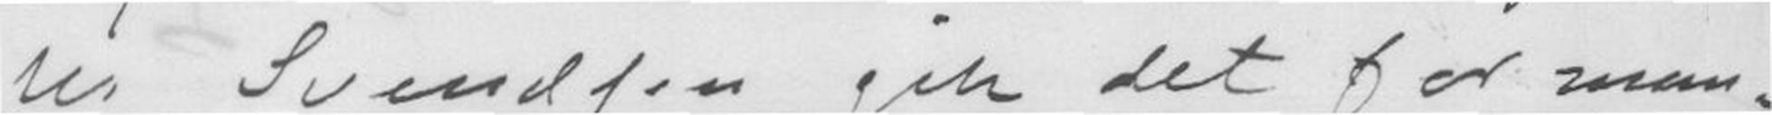hr Svendsen gik det for man-
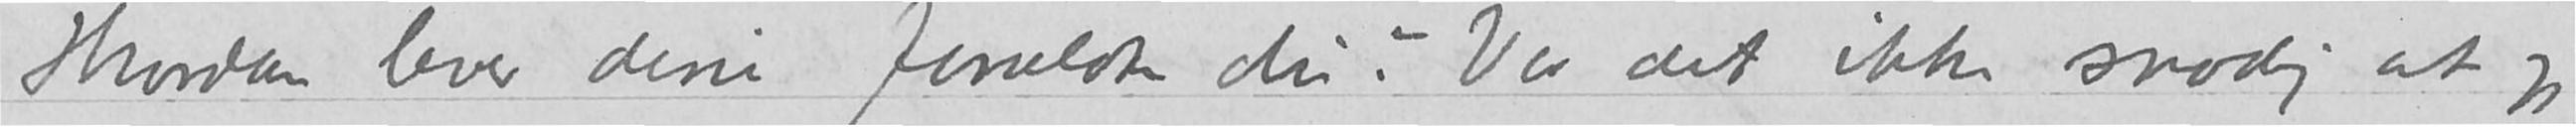Hvordan lever dine foreldre du? Var det ikke snodig at jeg
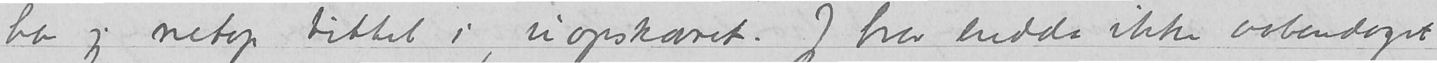har jeg netop tittet i, uopskaaret. Jeg har endda ikke aabendaget
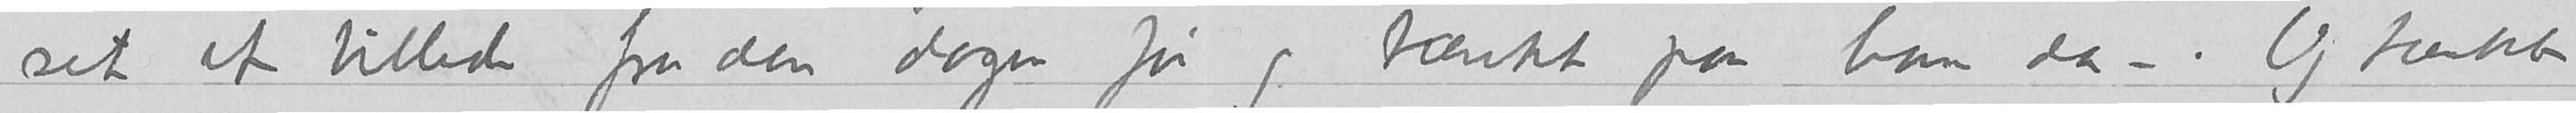set et billede fra den dagen før og tænkt paa ham da –. Vi tænkte
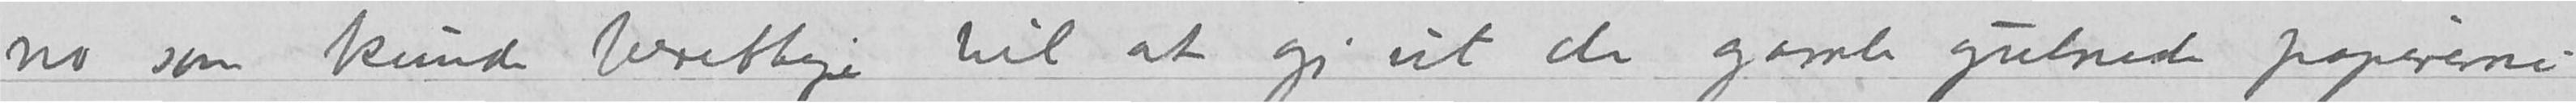no som kunde berettige til at gi ut de gamle gulnede papirerne.
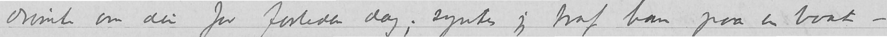drømte om din far forleden dag; syntes jeg traf ham paa en baat –
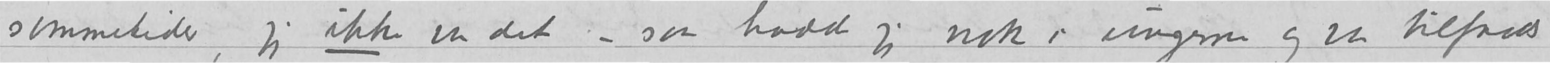sommetider, jeg ikke var det – saa hadde jeg nok i ungerne og var tilfreds med
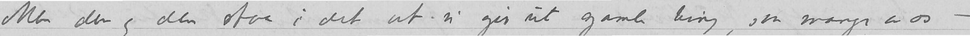Men den og den staar i det at vi gir ut gamle ting, saa mange av os –
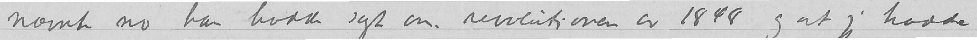nævnte no han hadde sagt om revolutionen av 1848 og at jeg hadde
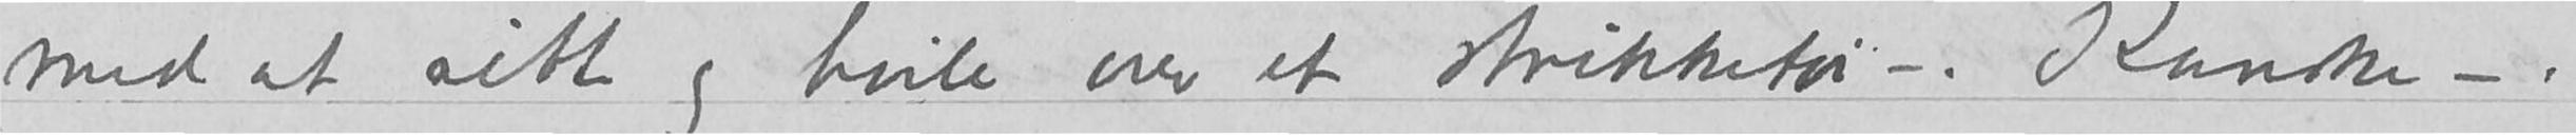at sitte og hvile ut over et strikketøi –. Kanske –.
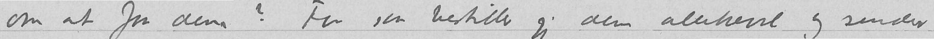om at faa dem? For saa bestiller jeg dem allikevel og sender
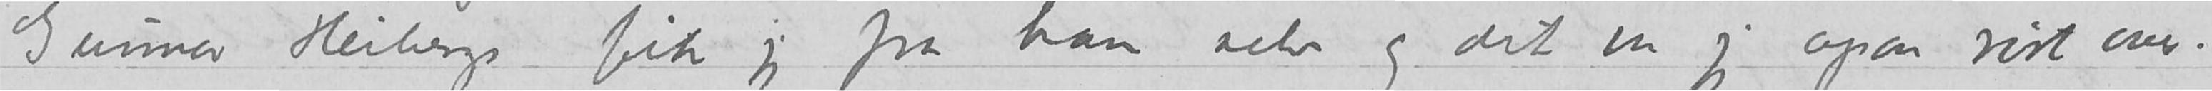Gunnar Heibergs fik jeg fra ham selv og det var jeg ogsaa rørt over.
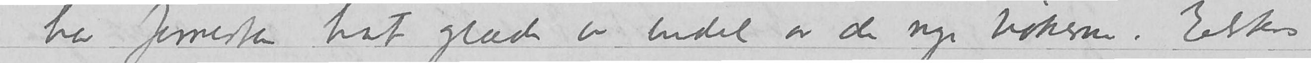Jeg har forresten hat glæde av endel av de nye bøkerne. Elsters
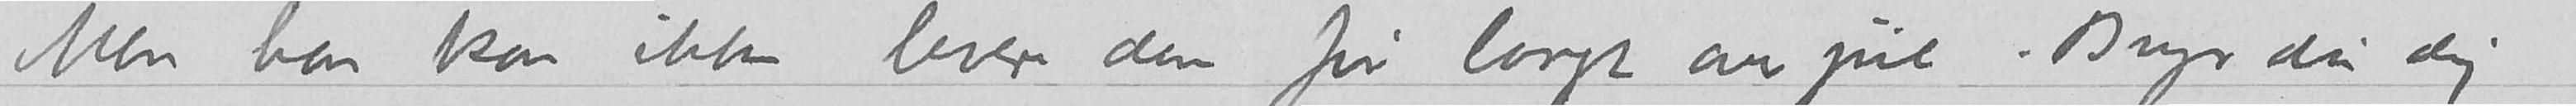Men han kan ikke levere dem før langt over jul. Bryr du dig
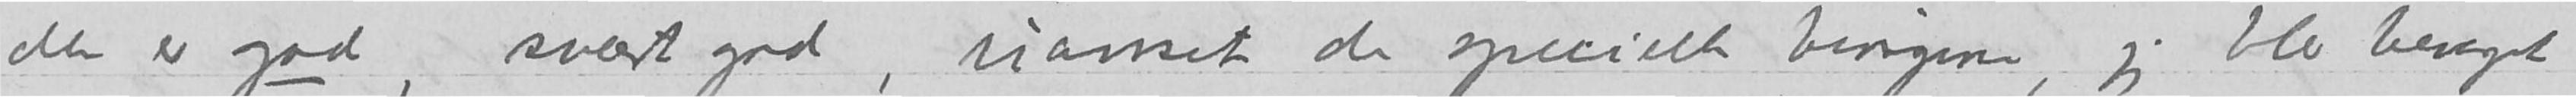den er god, svært god, uanset de specielle tingene, jeg ble bevæget
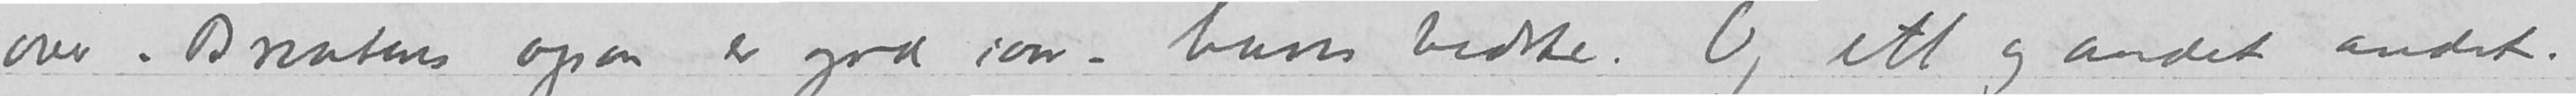over. Braatens ogsaa er god iaar – hans bedste. Og ett og andet andet.
